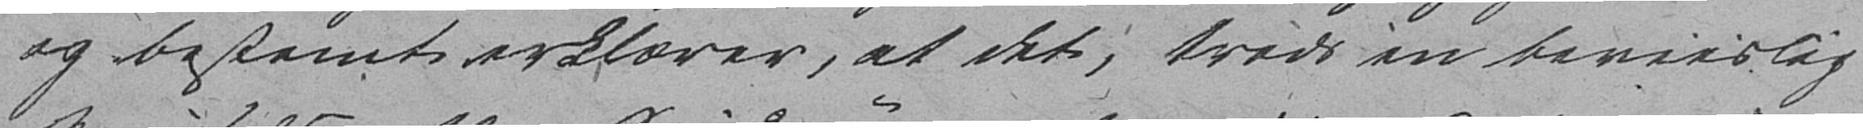og bestemt erklærer, at det, trods en beviislig
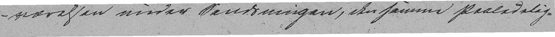værelsen under Sandsningen, den samme Paaledelig-
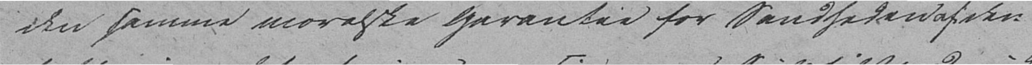den samme moralske Garantie for Sandheden af den
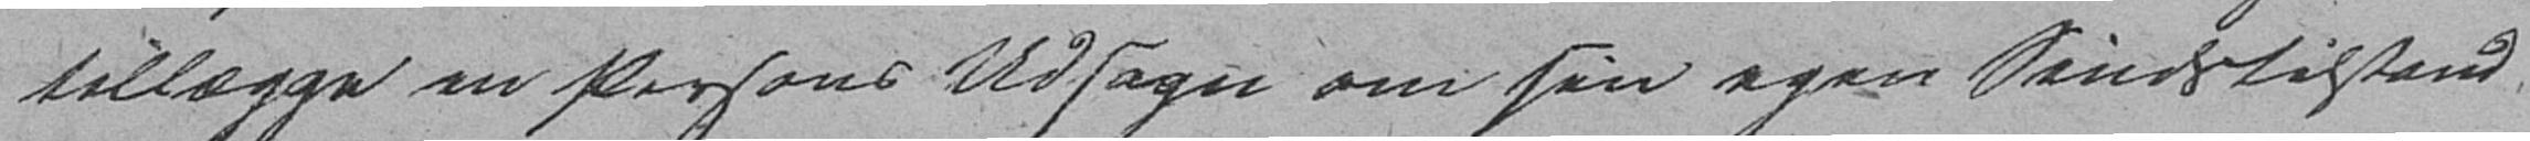tillægge en Persons Udsagn om sin egen Sindstilstand
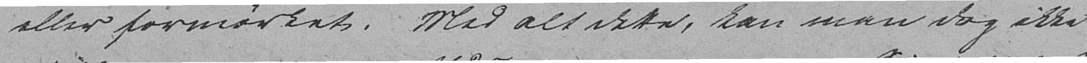eller formørket. Med alt dette, kan man dog ikke
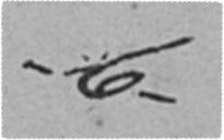- 6 -
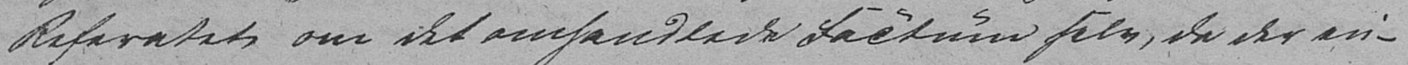Referatet om det omhandlede Factum selv, da der in-
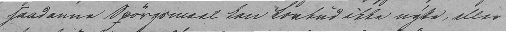saadanne Spørgsmaal kan Lovbud ikke nytte, eller
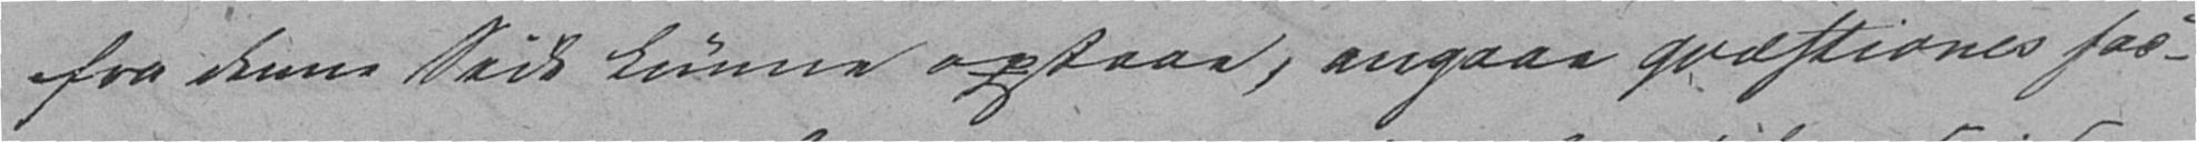fra denne Side kunne opstaae, angaar qvæstiones fac-
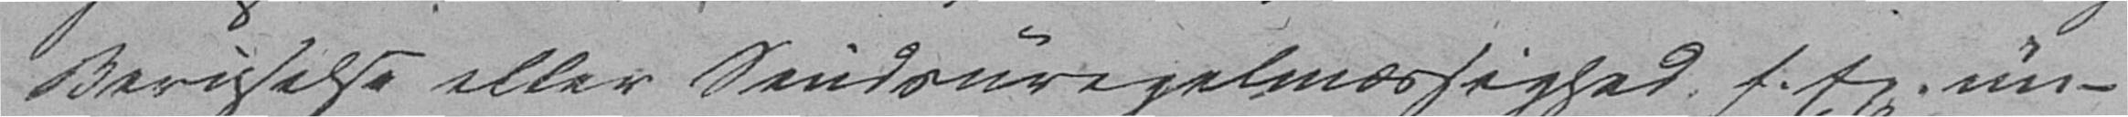Beruselse eller Sindsuregelmæssighed f. Ex. un-
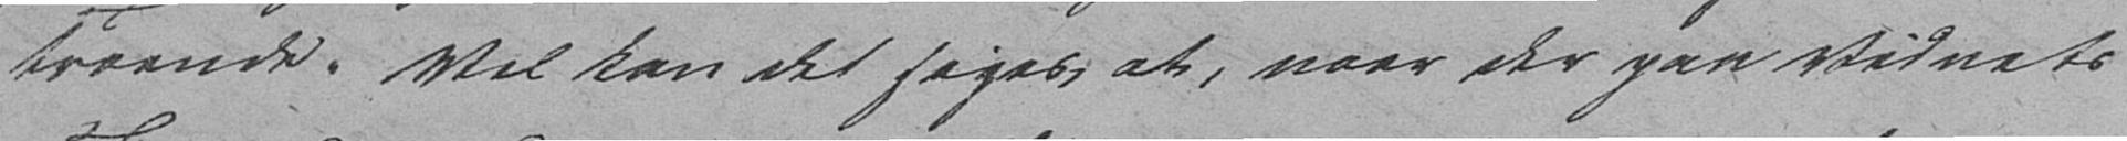Troende. Vel kan det siges, at, naar der paa Vidnets
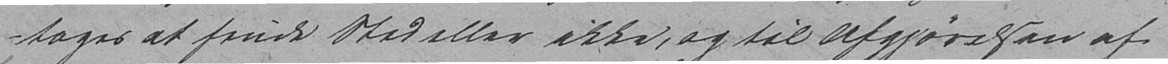tages at finde Sted eller ikke, og til Afgjørelsen af
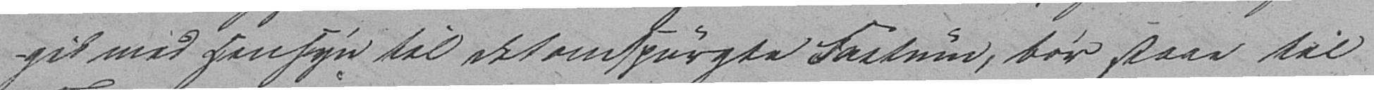gik med Hensyn til det omspurgte Factum, bør staae til
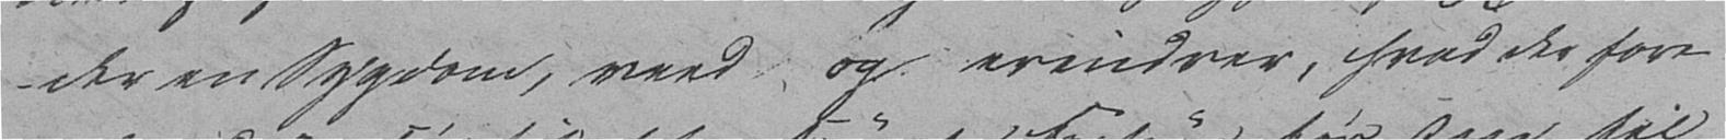der en Sygdom, veed og erindrer, hvad der fore-
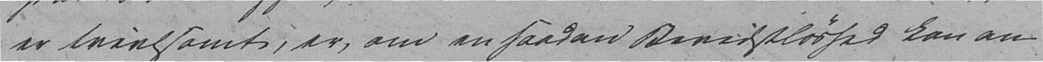er tvivlsomt, er, om en saadan Bevidstløshed kan an-
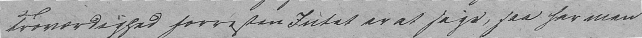Troværdighed forresten Intet er at sige, saa har man
